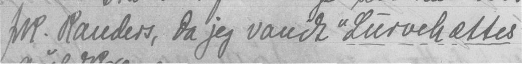frk. Randers, da jeg vandt "Lurvehættes"
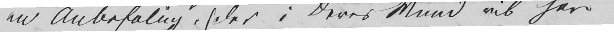en Anbefaling, (der i Deres Mund vil have
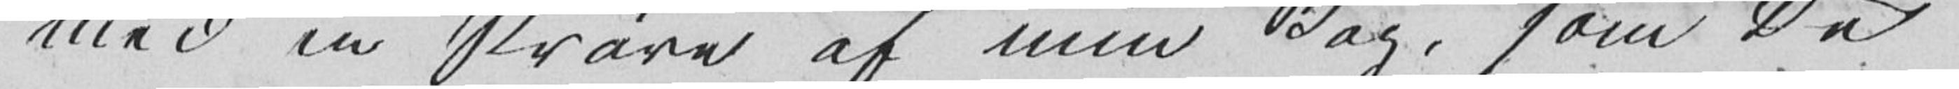med en Prøve af min Bog, som De
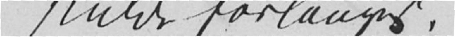skulde forlanges.
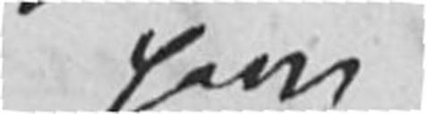paa
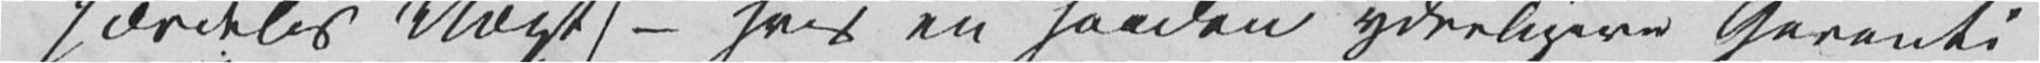særdeles Vægt) – hvis en saadan yderligere Garanti
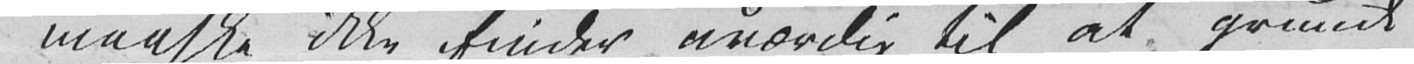maaske ikke finder uværdig til at grunde
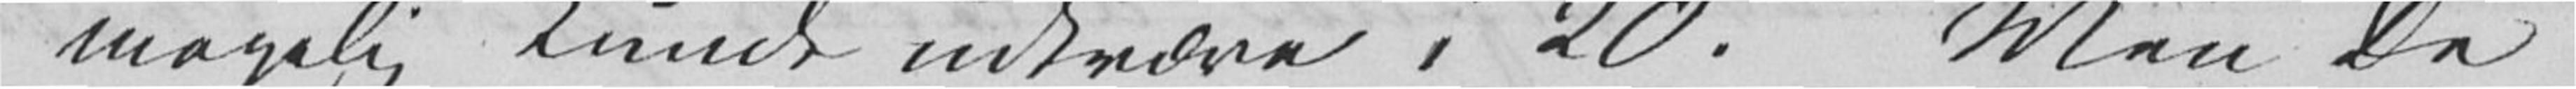magelig kunde udtvære i 20. Men De
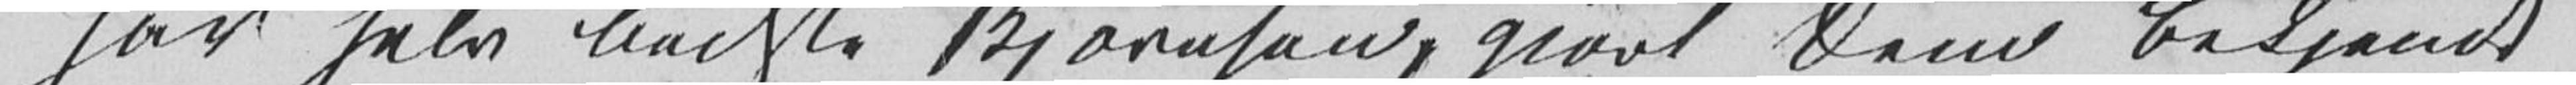har selv bedste Bjørnson, gjort Dem bekjendt
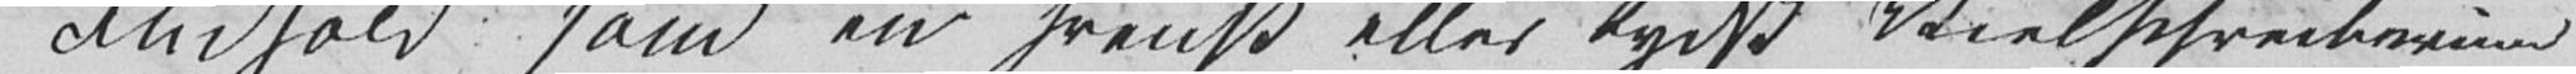Indhold som en svensk eller tydsk Vielschreiberinne
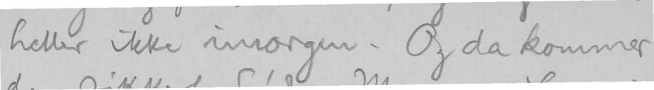heller ikke imorgen. Og da kommer
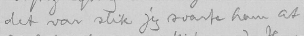det var slik jeg svarte ham at
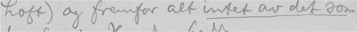Loft) og fremfor alt intet av det som
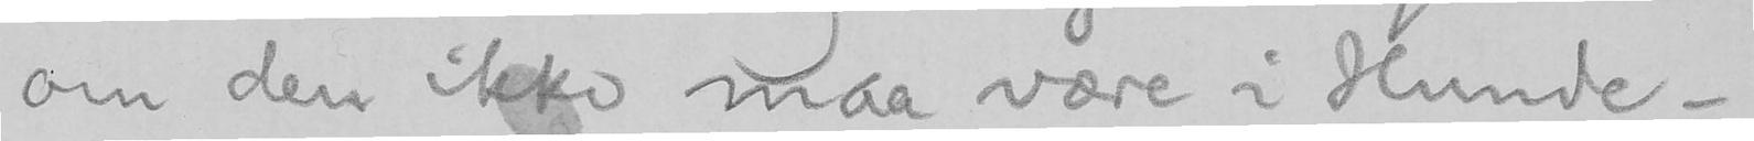om den ikke maa være i Hunde-
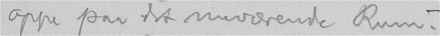oppe paa det nuværende Rum.
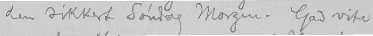den sikkert Søndag Morgen. Gad vite
